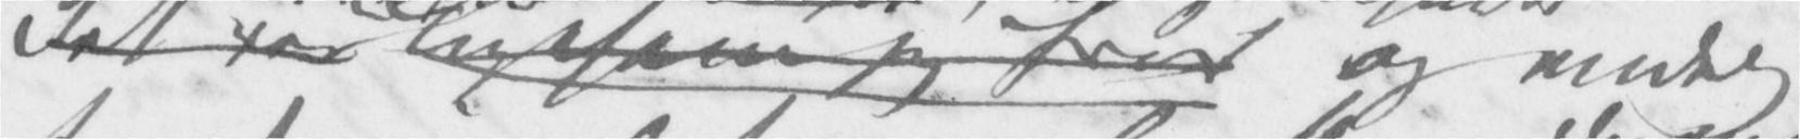det var ligesom jeg frøs og endog
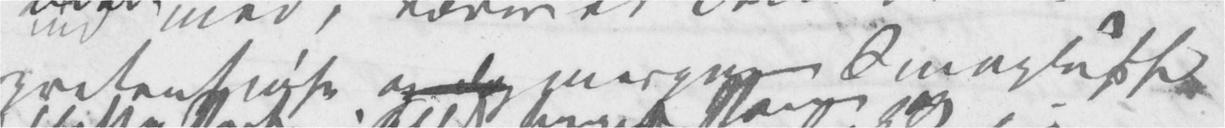pretentiøse og dog marqu Smagløshed
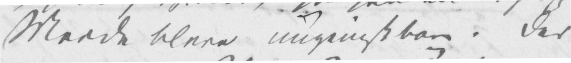Maade bleve ungenissbare. Der
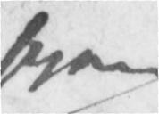<...>
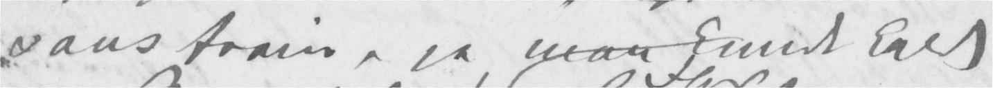sans frein, ja man kunde kaldt
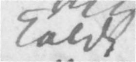kalde
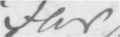Flor
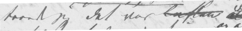troede jeg det var Luften
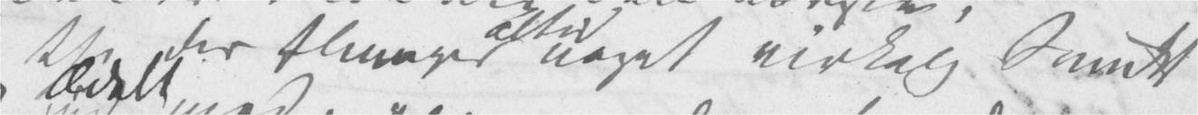thi der slumper  noget virkelig Smukt
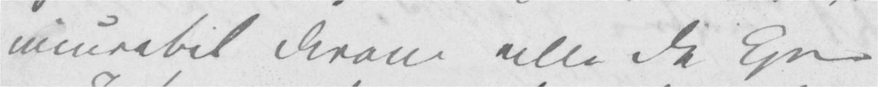incurabel derom ville De kjere
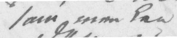som man kan
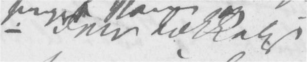– denne tækkelige
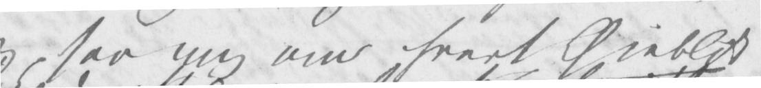Jeg saa mig om hvert Øjeblik
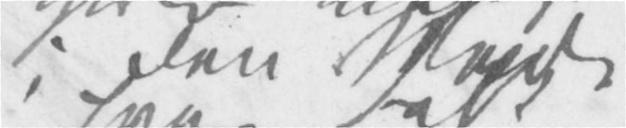i den Maade
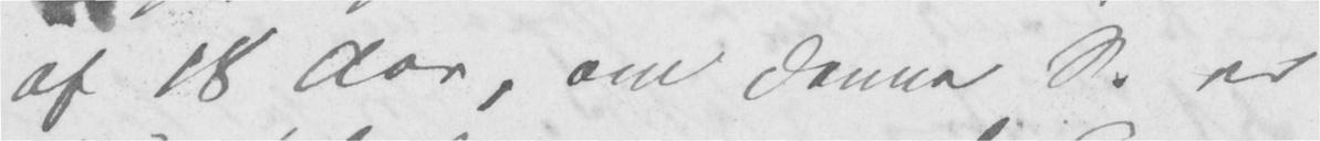af 18 Aar, om denne S. er
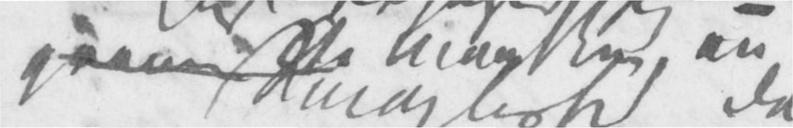<...>
